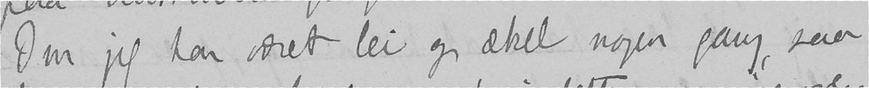Om jeg har været lei og ækel nogen gang, saa
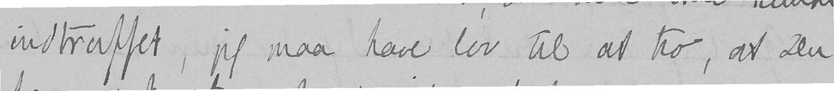indtruffet, jeg maa have lov til at tro, at Du
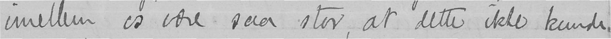imellem os være saa stor, at dette ikke kunde
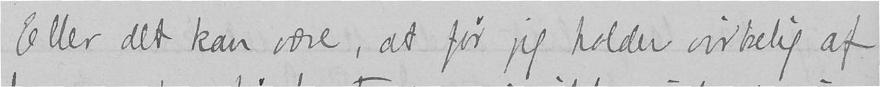Eller det kan være, at før jeg holder virkelig af
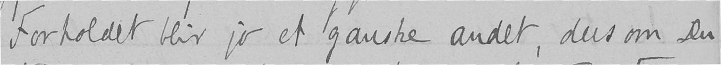Forholdet blir jo et ganske andet, dersom Du
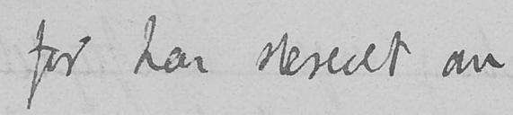for har skrevet om
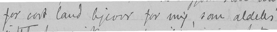for vort land ligeover for mig, som aldeles
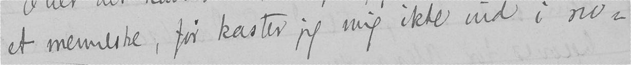et menneske, før kaster jeg mig ikke ind i riv-
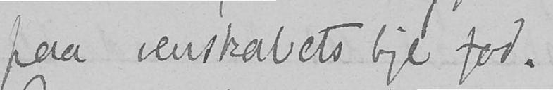paa venskabets lige fod.
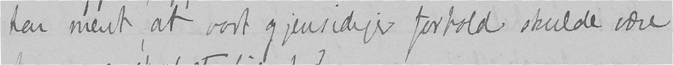har ment at vort gjensidige forhold skulde være
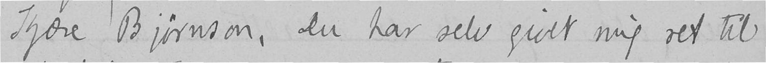Kjære Bjørnson, Du har selv givet mig ret til
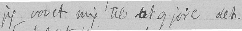jeg vovet mig til at gjøre det.
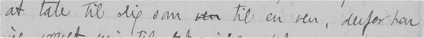at tale til Dig som ven til en ven, derfor har
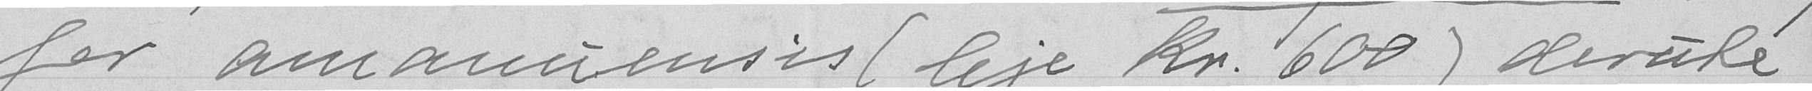for amanuensis (leje Kr. 600) derute
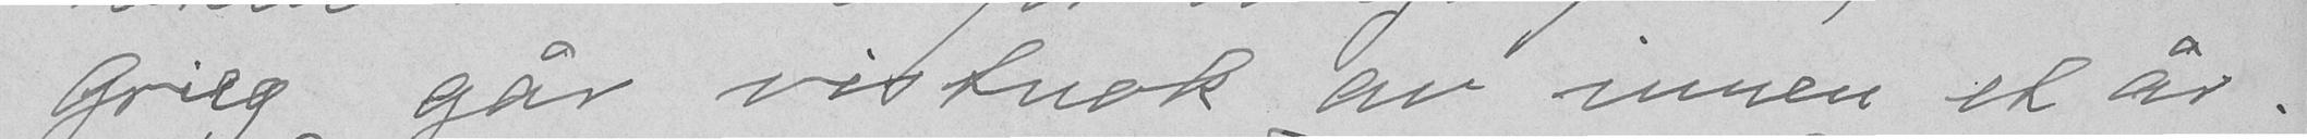Grieg går vistnok av innen et år.
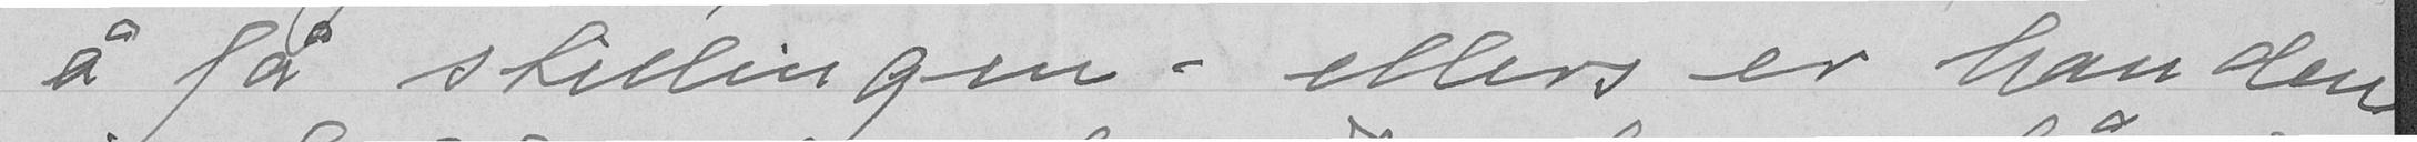å få stillingen - ellers er han den
